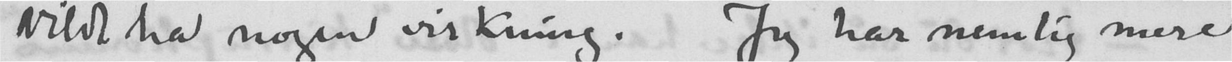vilde ha nogen virkning. Jeg har nemlig mere
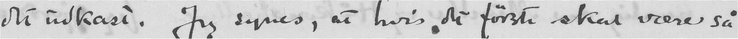det udkast. Jeg synes, at hvis det første skal være så
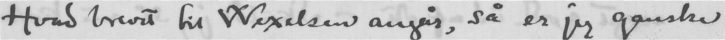Hvad brevet til Wexelsen angår, så er jeg ganske
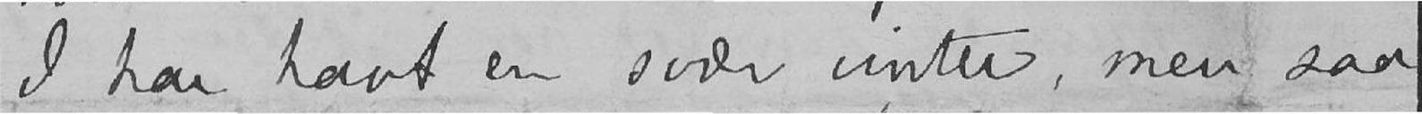I har havt en svær vinter, men saa
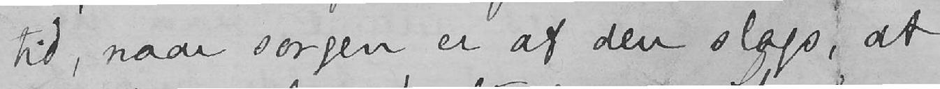tid, naar sorgen er af den slags, at
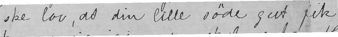ske lov, at din lille søde gut fik
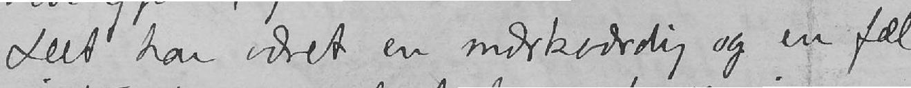Det har været en mærkværdig og en fæl
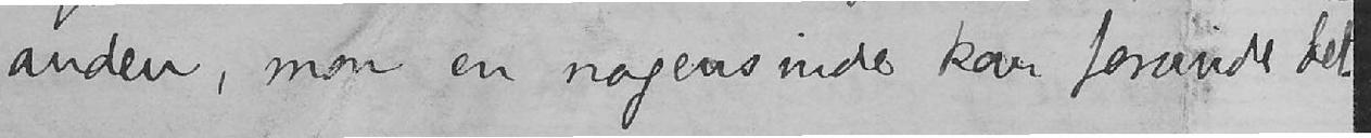anden, mon en nogensinde kan forandre det.
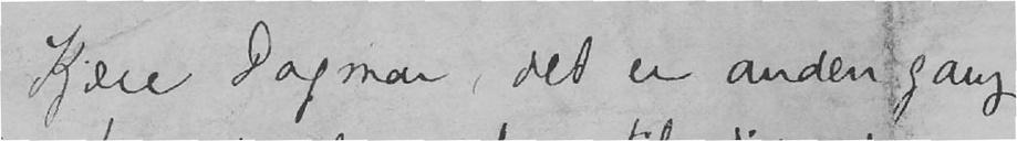Kjære Dagmar, det er anden gang
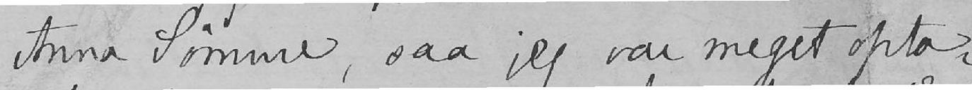Anna Sømme, saa jeg var meget opta-
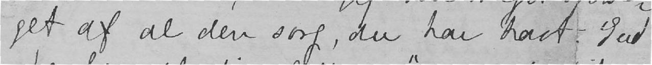get af al den sorg, du har havt. Gud
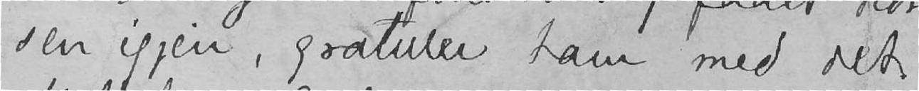sen igjen, gratuler ham med det.
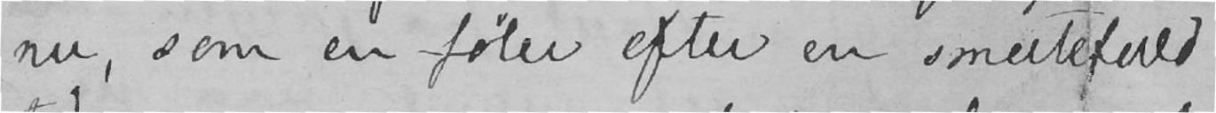nu, som en føler efter en smertefuld
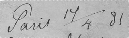Paris 17/4 81
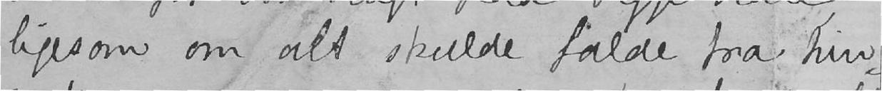ligesom om alt skulde falde fra hin-
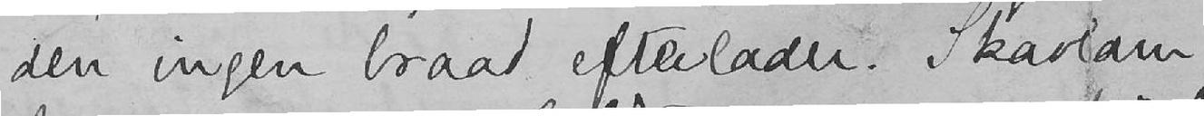den ingen braad efterlader. Skavlam
